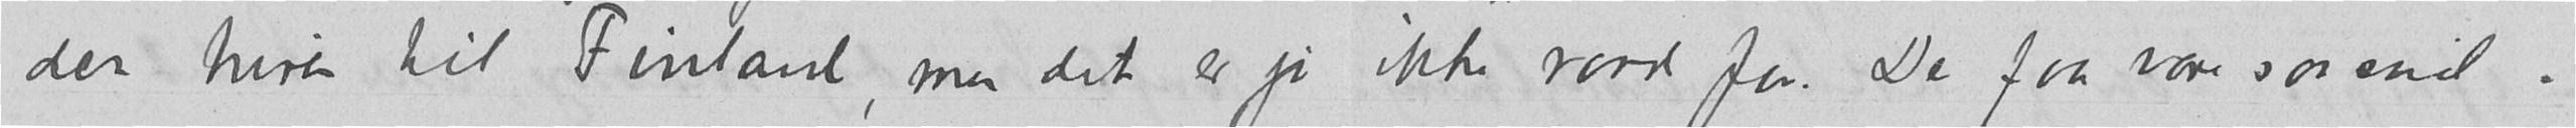den turen til Finland, men det er jo ikke raad for. De faar være saa snil
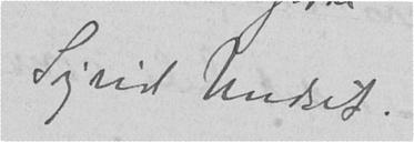Sigrid Undset.
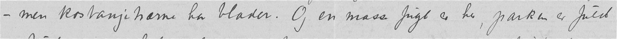- men kastanjetrærne har blader. Og en masse fugl er her, parken er fuld
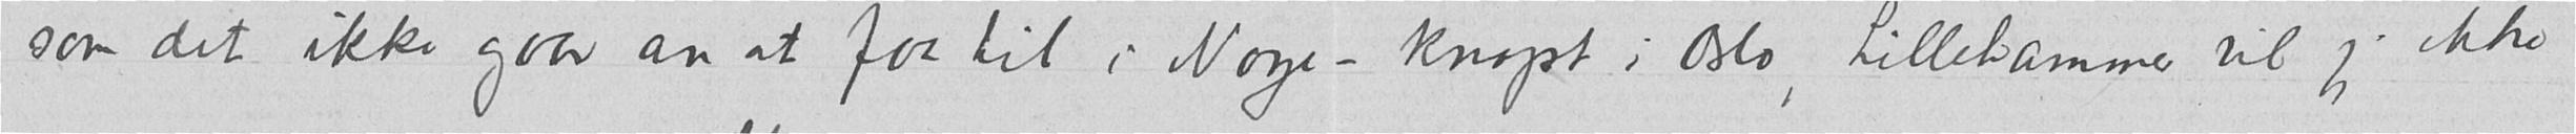som det ikke gaar an at faa til i Norge - knapt i Oslo, Lillehammer vil jeg ikke
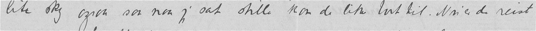lite sky ogsaa saa naar jeg sat stille kom de like bort til. Nu er de reist
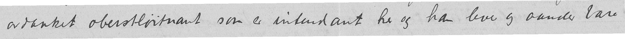avdanket oberstløitnant som er intendant her og han lever og aander bare
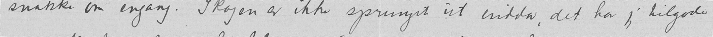snakke om engang. Skogen er ikke sprunget ut endda, det har jeg tilgode
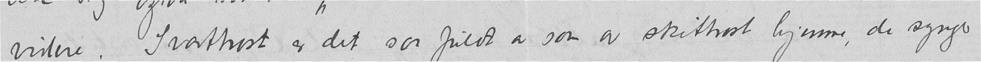videre. Svarttrost er det saa fuldt av som av skittrost hjemme, de synger
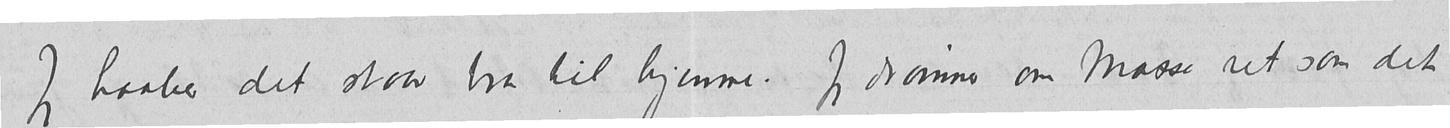Jeg haaber det staar bra til hjemme. Jeg drømmer om Mosse ret som det
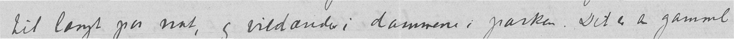til langt paa nat, og vildænder i dammene i parken. Det er en gammel
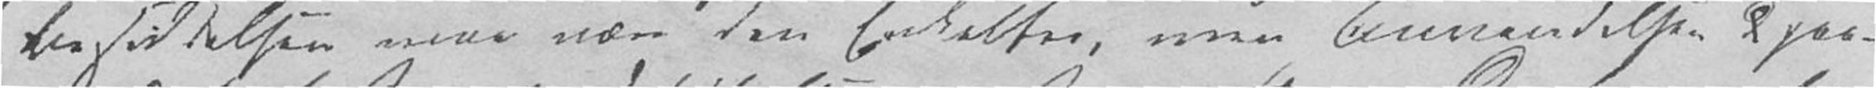Besiddelsen maa være den Enkeltes, men Anvendelse d paa-
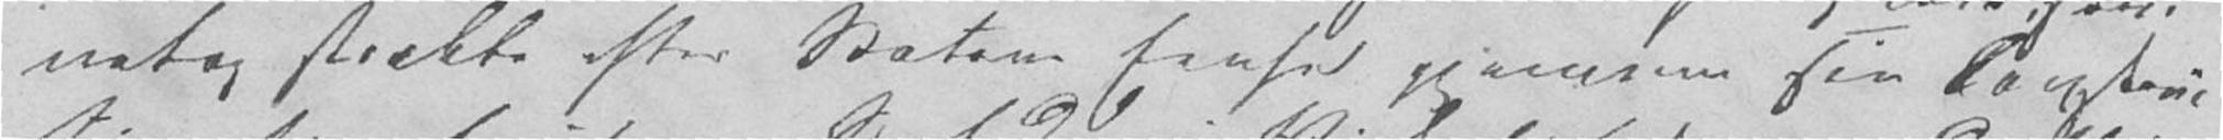netop stræbte efter Statens Eenhed gjennem sin Construc-
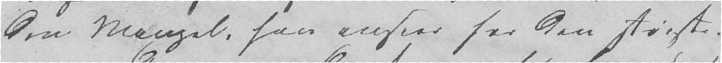den Mangel, han anseer for den største.
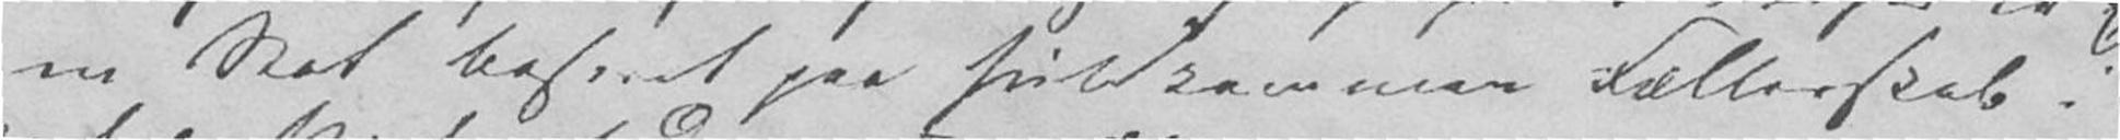en Stat baseret paa fuldkommen Fællesskab i
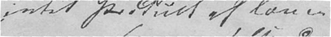intet Product af Loven.
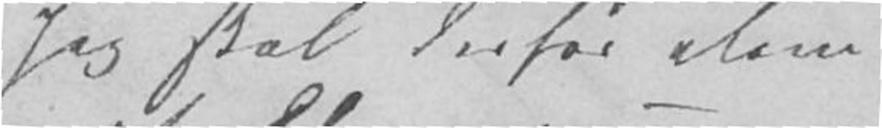Jeg skal derfor alene
